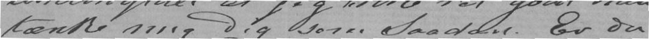tænke mig Dig som Saadan. Er du
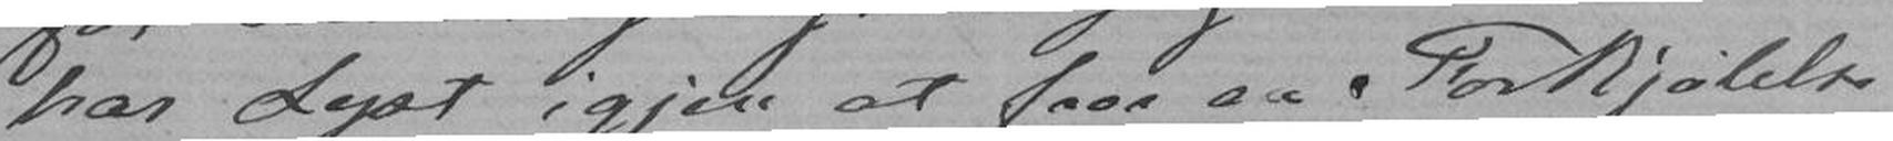har Lyst igjen at faae en Forkjølelse
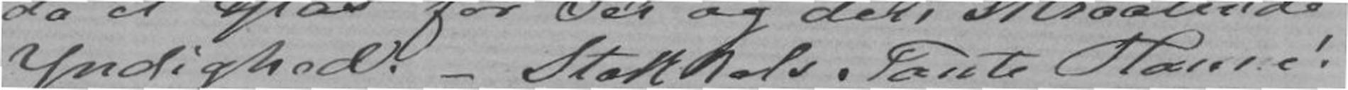Yndighed. - Stakkels Tante Hanne!
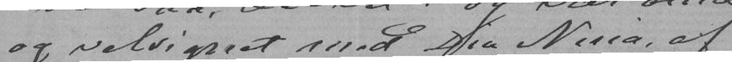og velsignet med Din Nina af
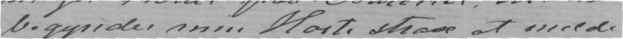begynder min Hoste strax at melde
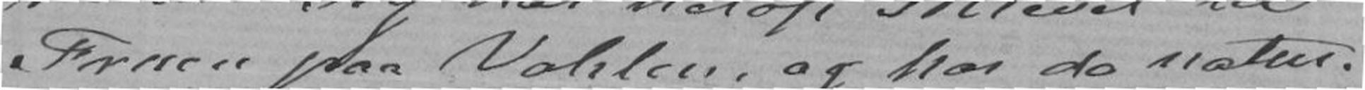Fruen paa Vahlen, og har da natur-
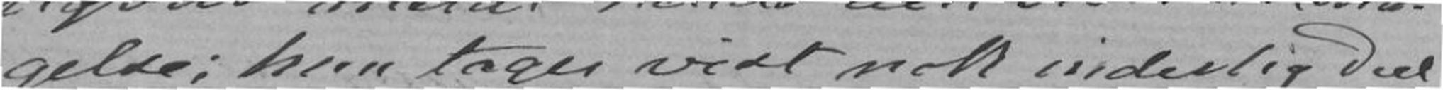gelse; hun tager vist nok inderlig Deel
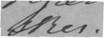sker.
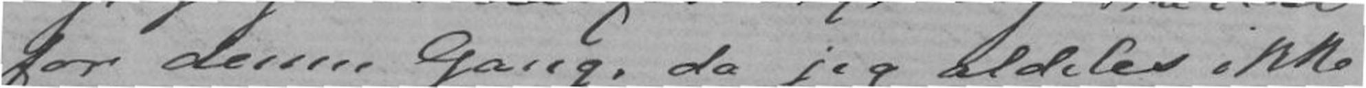for denne Gang, da jeg aldeles ikke
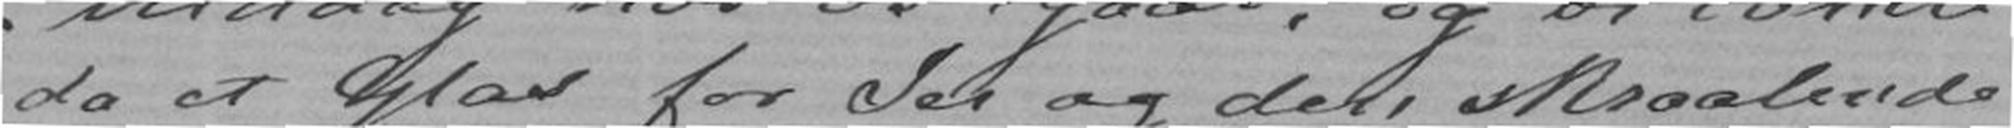da et Glas for Jer og den skraalende
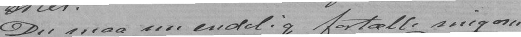Du maa nu endelig fortælle mig om
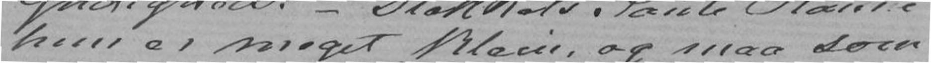hun er meget klein, og maa som
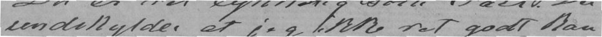undskylder at jeg ikke ret godt kan
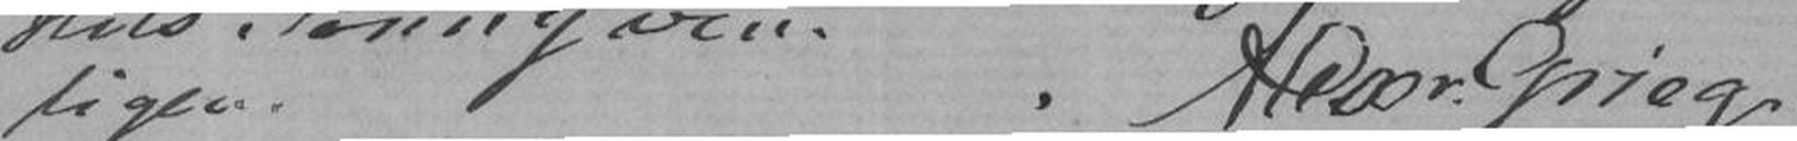ligen Alexr. Grieg
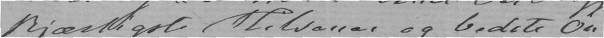kjærligste Hilsener og bedste Øn-
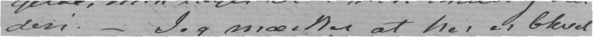deri. - Jeg mærker at her er blevet
